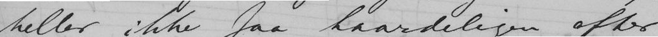heller ikke saa haardeligen efter
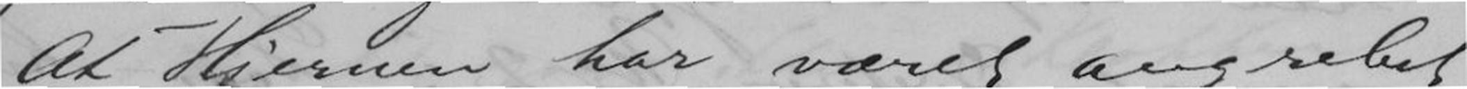At Hjernen har været angrebet
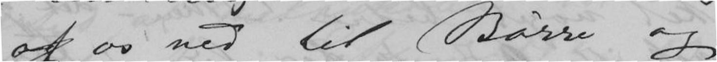jeg af os ned til Børre og
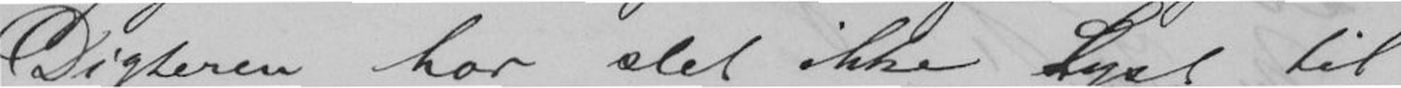Digteren har slet ikke Lyst til
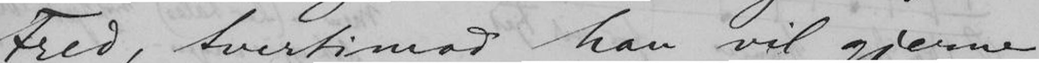Fred, tvertimod han vil gjerne
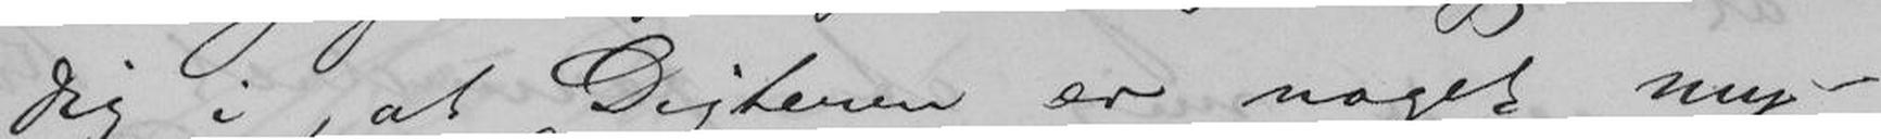Dig i, at Digteren er noget my-
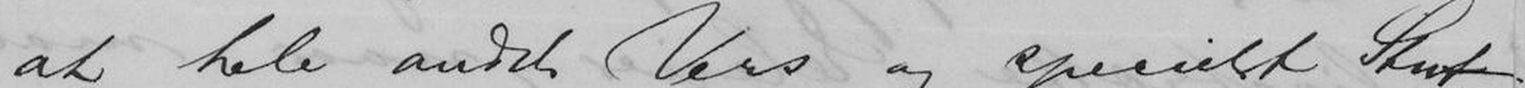at hele andet Vers og spesielt Slut-
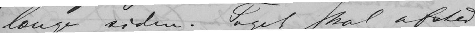længe siden. Toget skal afsted,
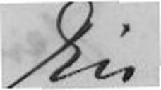Din
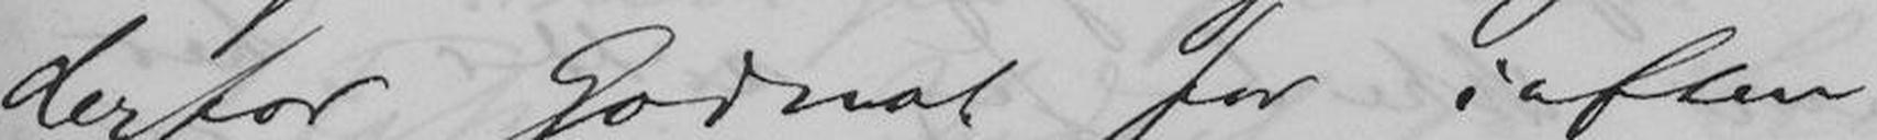derfor Godnat for iaften!
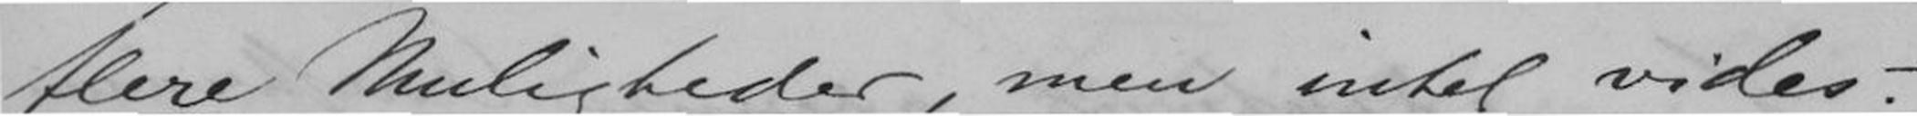flere Muligheder, men intet vides. -
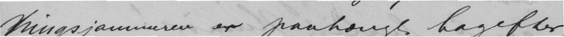ningsjammeren er paahengt bagefter.
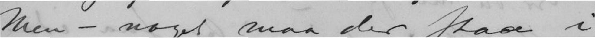Men - noget maa der staa i
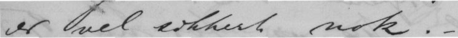er vel sikkert nok. -
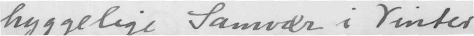hyggelige Samvær i Vinter,
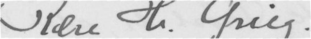Kære Hr. Grieg.
